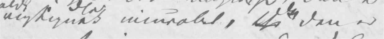rigtignok incurabel, thi da den er
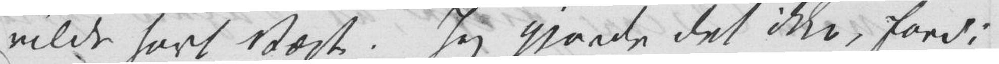vilde havt Vægt. Jeg gjorde det ikke, fordi
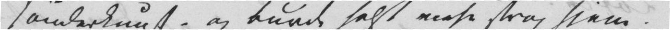sønderknust, og burde helst reise strax hjem.
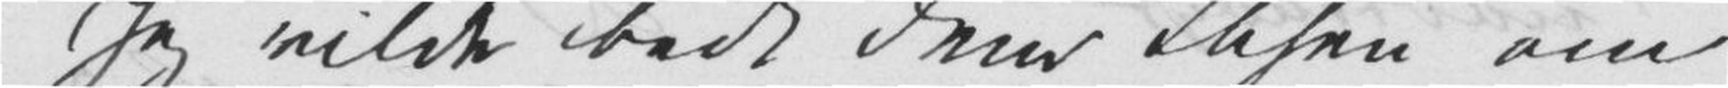Jeg vilde bede Dem Ibsen om
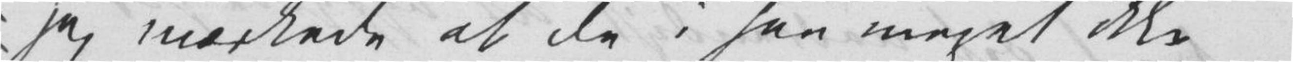jeg mærkede at de i saa meget ikke
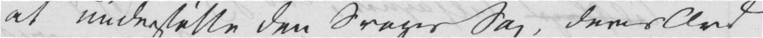at understøtte den Svages Sag, deres Ord
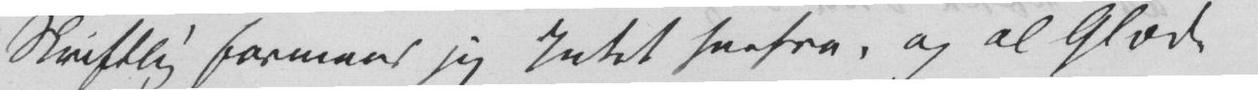Skriftlig formaar jeg Intet herfra, og al Glæde
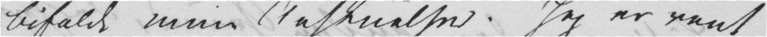bifalde min Anskuelse. Jeg er rent
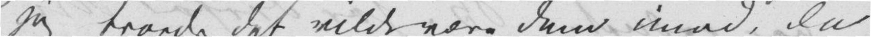jeg troede det vilde være Dem imod, da
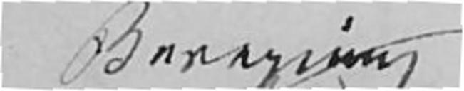Beregning
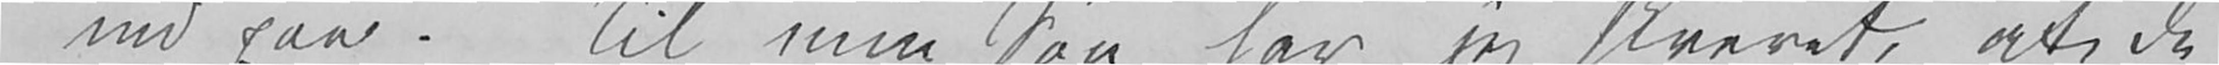ind paa. Til min Søn har jeg skrevet, at de
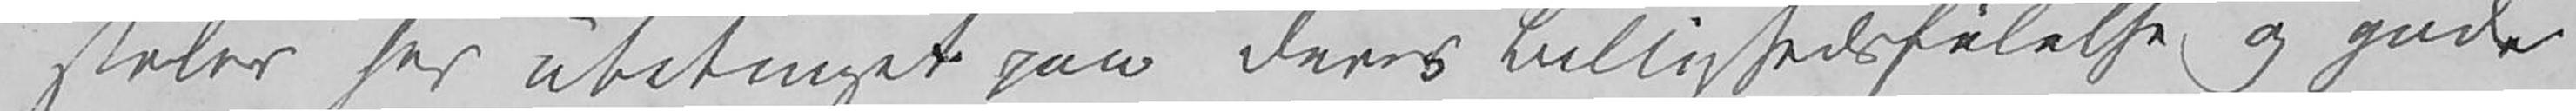stoler her ubetinget paa Deres Billighedsfølelse og gode
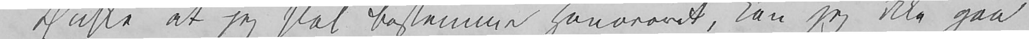Ønske at jeg skal bestemme Honoraret, kan jeg ikke gaa
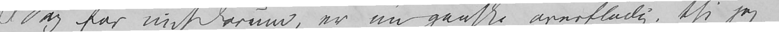Sag for mit For um, er nu ganske overflødig, thi jeg
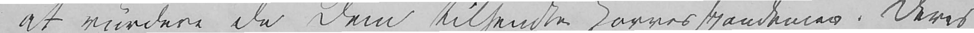at vurdere de Dem tilsendte Korresspondencer. Deres
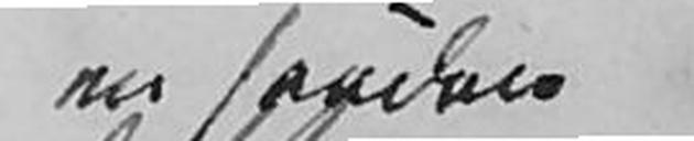en saadan
